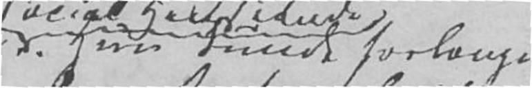. Hun kunde forlange
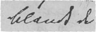blandt de
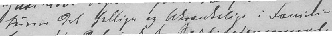tuerer det hellige og ukrænkelige i Familie-
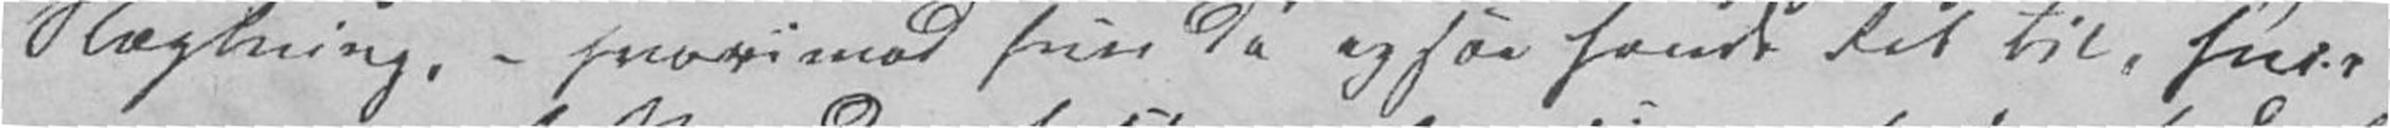Slægtning, – hvorimod hun da ogsaa havde Ret til, hvis
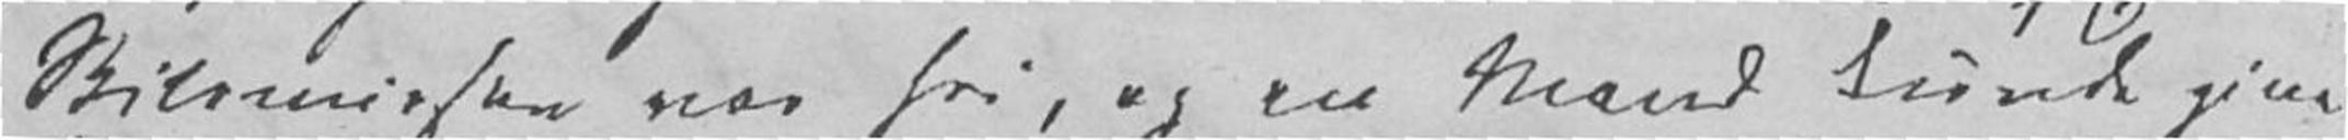Skilsmissen var fri, og en Mand kunde give
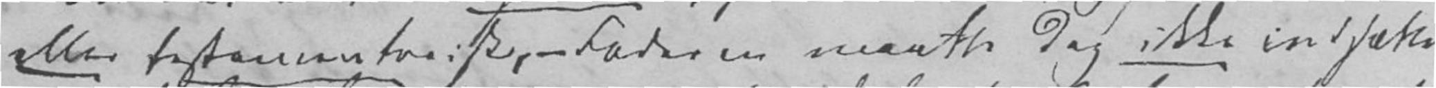eller testamentarisk, - Faderen maatte dog ikke indsætte
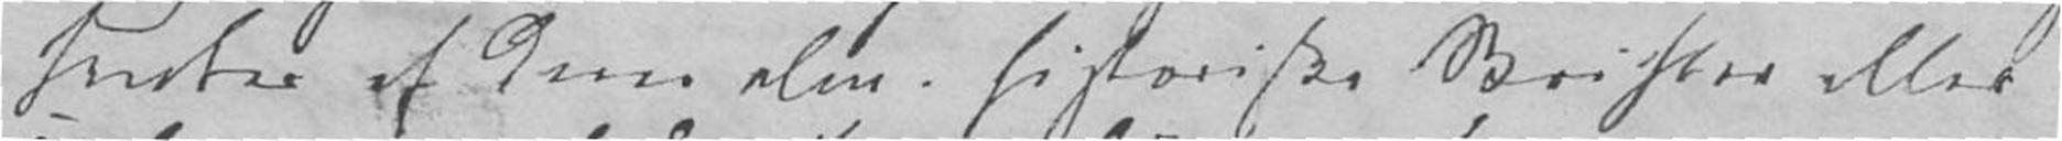hentes af deres alm. historiske Skrifter eller
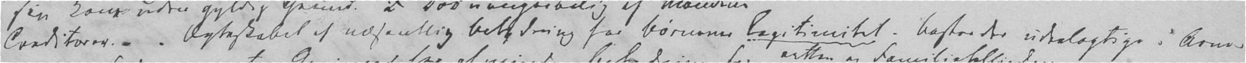Creditorer.- Ægteskabet af væsentlig Betydning for Børnenes Legitimitet. Bastarder udeelagtige i Arve-
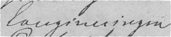Lovgivningen
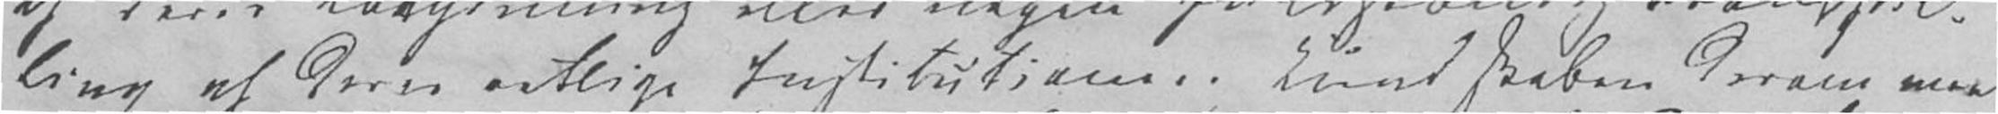ling af deres retlige Institutioner. Kundskaben derom maa
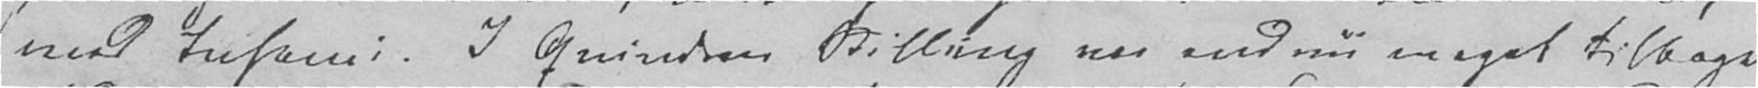med Infami. I Qvindens Stilling var endnu meget tilbage
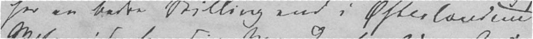her en bedre Stilling end i Østerlandene
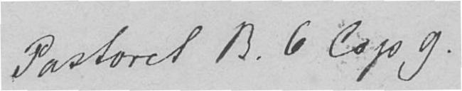Pastorel B. 6 Cap 9.
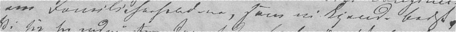om Familieforholdene, som vi kjende Bedst.
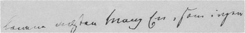Lovene næsten tvang En, som ingen
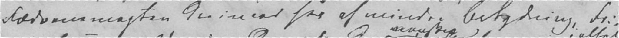Fædrenemagten derimod her af mindre Betydning, Fri-
